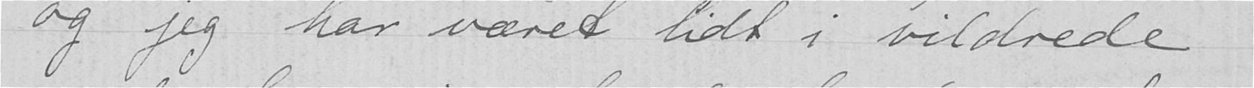og jeg har været lidt i vildrede
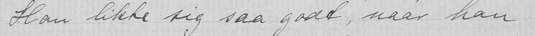Han likte sig saa godt, naar han
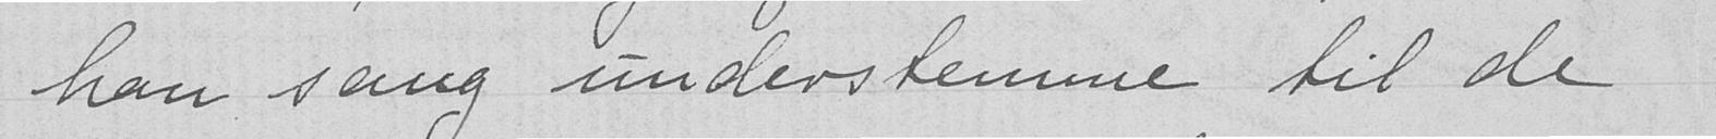han sang understemme til de
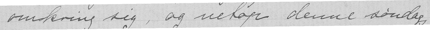omkring sig, og netop denne søndags-
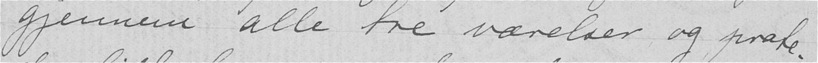gjennem alle tre værelser, og prate-
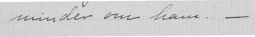minder om ham. - - -
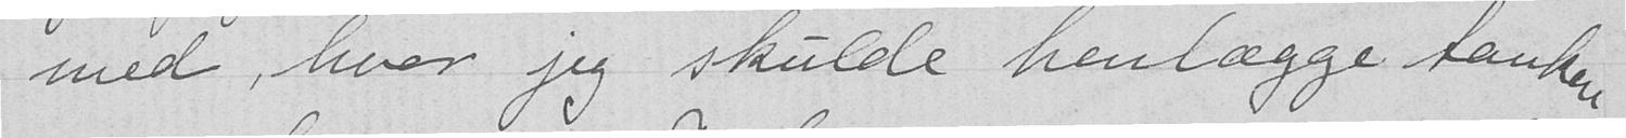med, hvor jeg skulde henlægge tankene
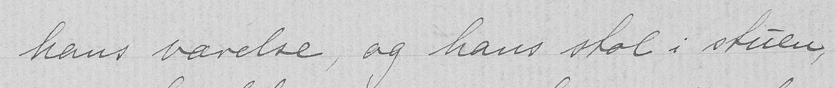hans værelse, og hans stol i stuen,
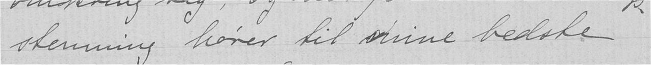stemning hører til mine bedste
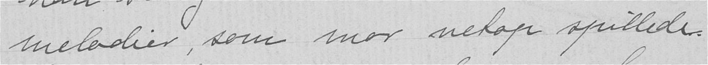melodier, som mor netop spillede.
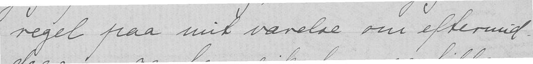regel paa mit værelse om eftermid-
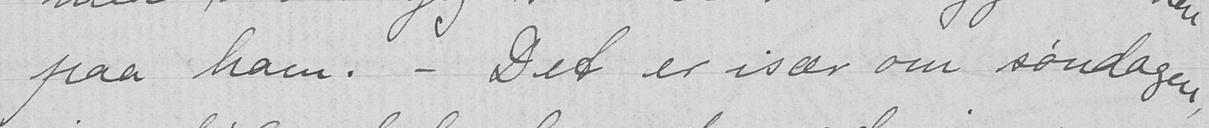paa ham. - Det er især om søndagen,
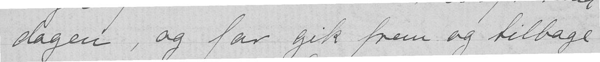dagen, og far gik frem og tilbage
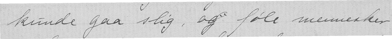kunde gaa slig, og føle mennesker
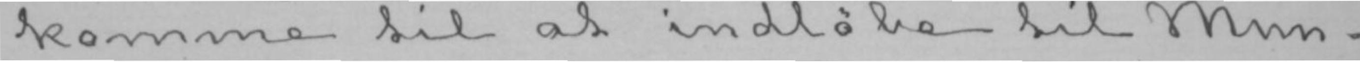komme til at indløpe i Mün-
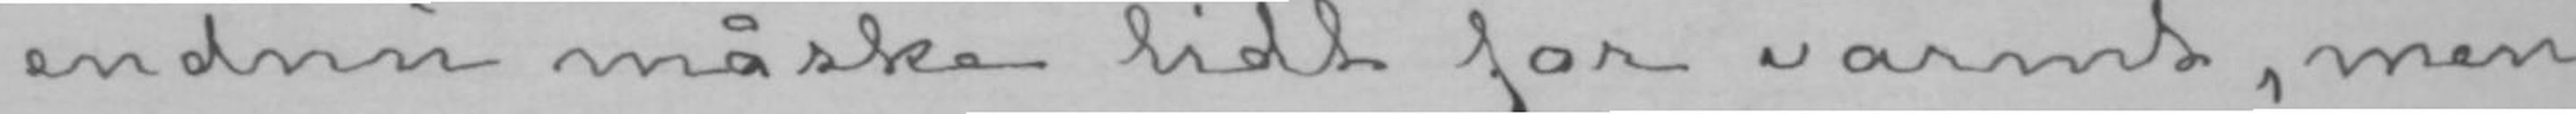endnu måske lidt for varmt, men
